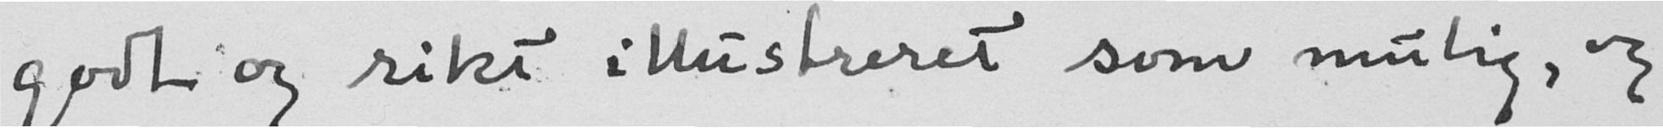godt og rikt illustreret som mulig, og
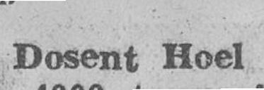Dosent Hoel
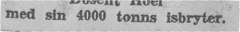med sin 4000 tonns isbryter.
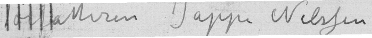Forfatteren Jappe Nilssen
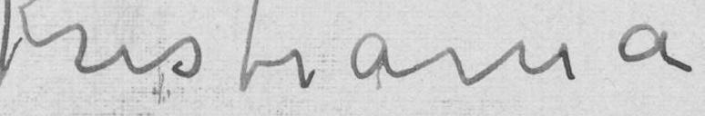Kristiania
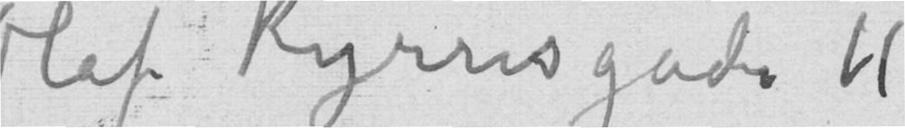Olaf Kyrresgade 11
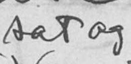sat og
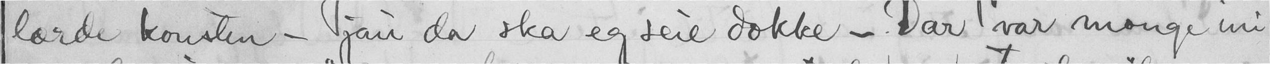lærde konsten - jau da ska eg seie dokke - Dar var monge ini
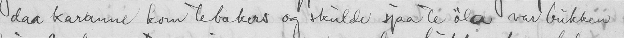daa karanne kom tebakers og skulde sjaa te øla var bukken
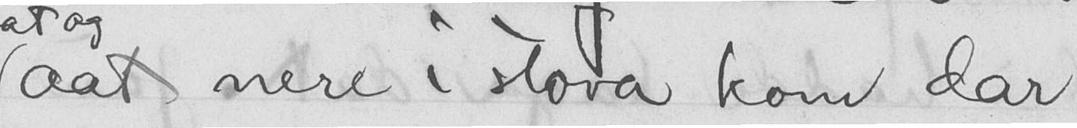aat nere i stova kom dar
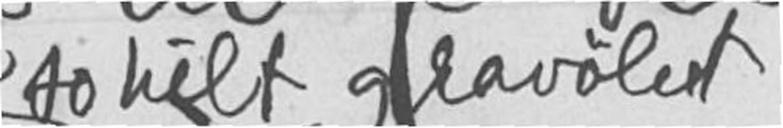so hilt gravølet
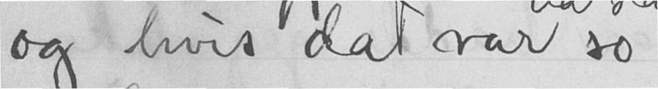og hvis dat var so
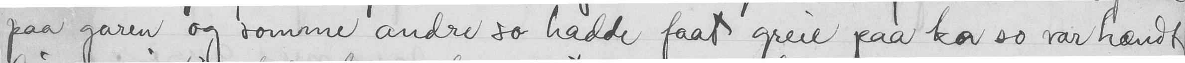paa garen og somme andre so hadde faat greie paa ka so var hændt
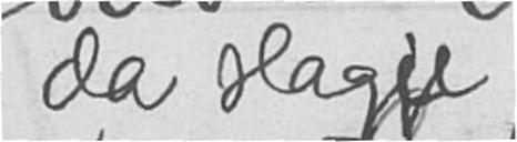da slagte
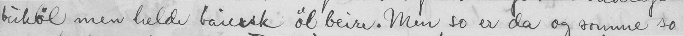bukøl men helde baiersk øl beire. Men so er da os somme so
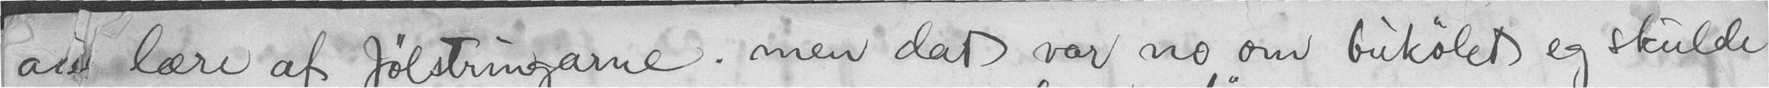at lære af Jølstringarne, men dat var no om bukølet eg skulde
